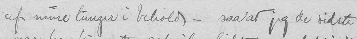af mine lunger i behold - saa at jeg de sidste
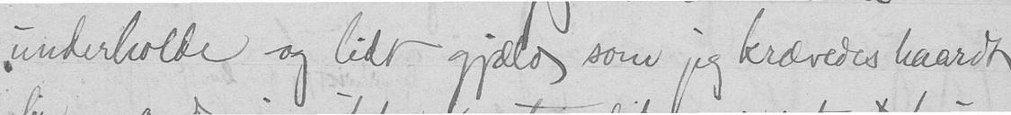underholde og lidt gjæld som jeg krævedes haardt
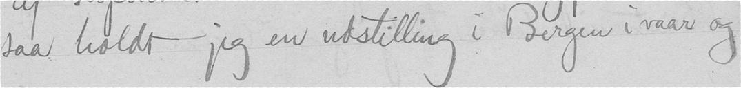saa holdt jeg en udstilling i Bergen i vaar og
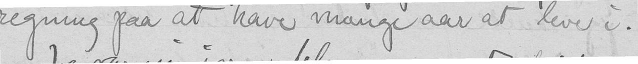regning paa at have mange aar at leve i.
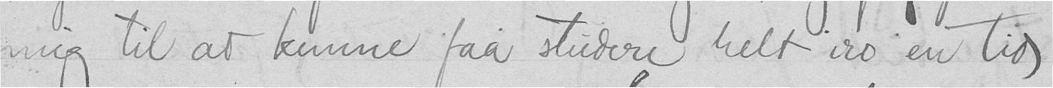mig til at kunne faa studere helt iro en tid
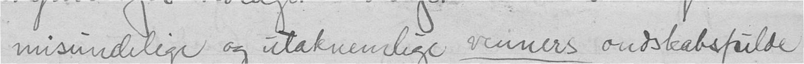misundelige og utaknemlige venners ondskabsfulde
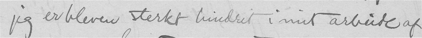jeg er bleven sterkt hindret i mit arbeide af
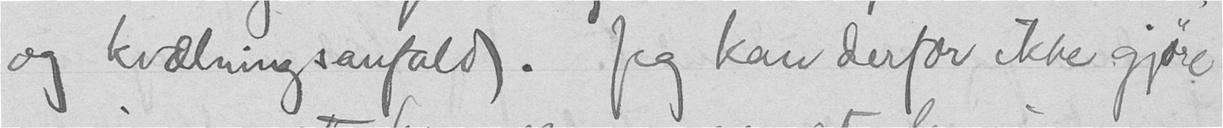og kvælningsanfald. Jeg kan derfor ikke gjøre
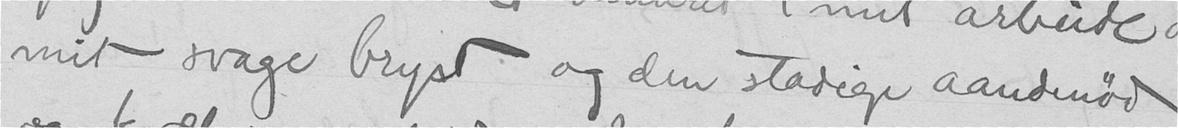mit svage bryst og den stadige aandenød
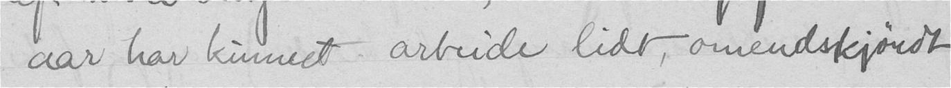aar har kunnet arbeide lidt, omendskjøndt
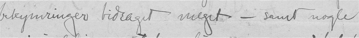bekymringer bidraget meget - samt nogle
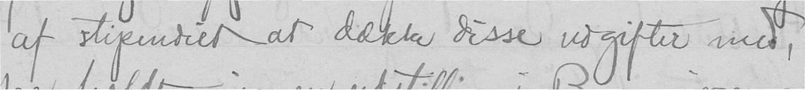af stipendiet at dække disse udgifter mod,
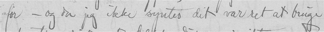for - og da jeg ikke syntes det var ret at bruge
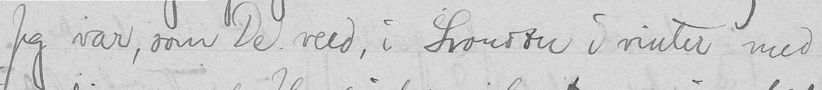Jeg var, som De veed, i London i vinter med
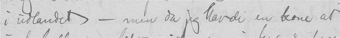i udlandet, - men da jeg havde en kone at
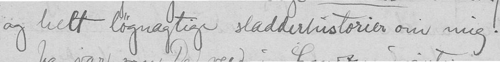og helt løgnagtige sladderhistorier om mig!
